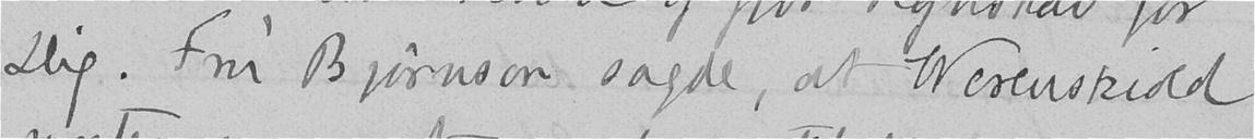Dig. Fru Bjørnson sagde, at Werenskiold
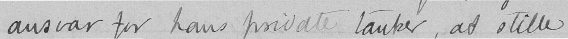ansvar for hans private tanker, at stille
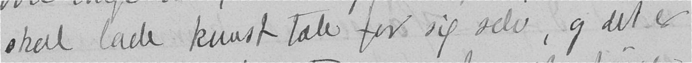skal lade kunst tale for sig selv, og det er
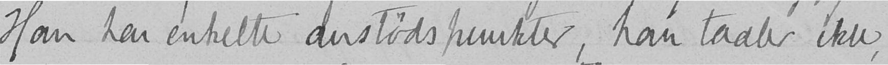Han har enkelte anstødspunkter, han taaler ikke,
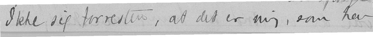Ikke sig forresten, at det er mig, som har
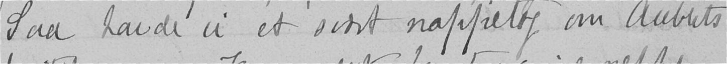Saa havde vi et svært nappetak om Auberts
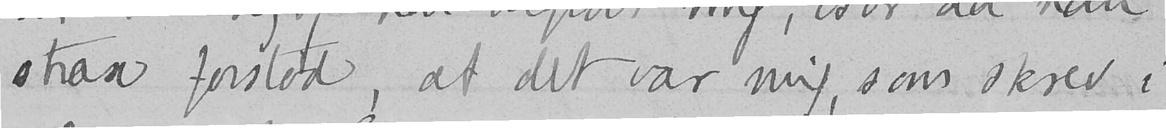strax forstod, at det var mig, som skrev i
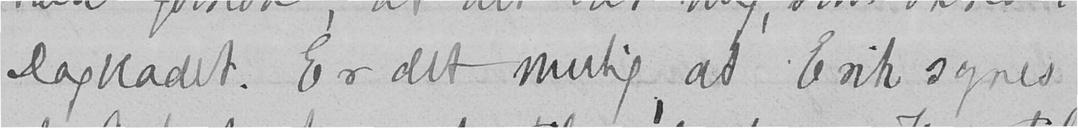Dagbladet. Er det mulig, at Erik synes
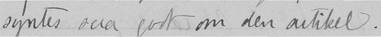syntes saa godt om den artikel.
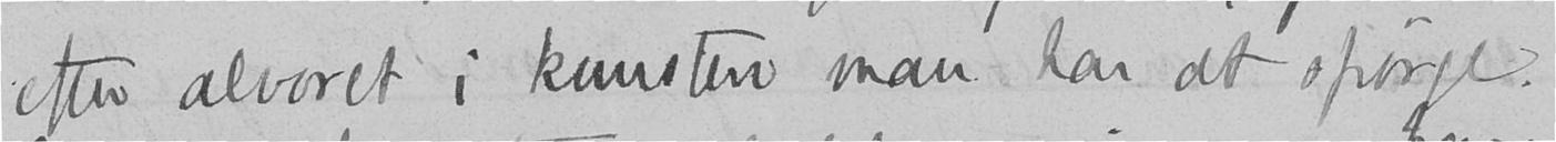efter alvoret i kunsten man har at spørge.
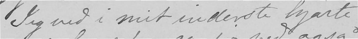Jeg ved i mit inderste hjærte
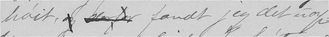høit, og derfor fandt jeg det uop-
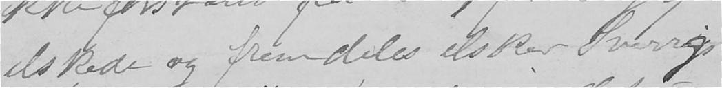elskede og fremdeles elsker Sverrig
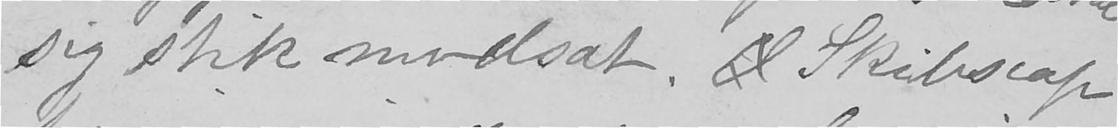sig stik modsat. Skibscap-
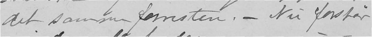det samme forresten. - Nu forstår
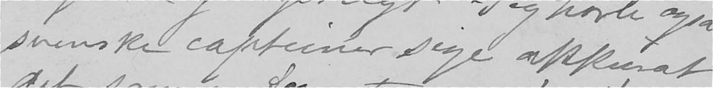svenske capteiner sige akkurat
